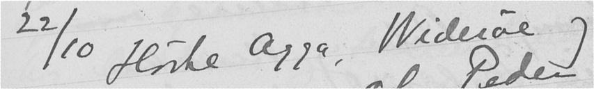22/10 Hørte Agga, Widerøe og
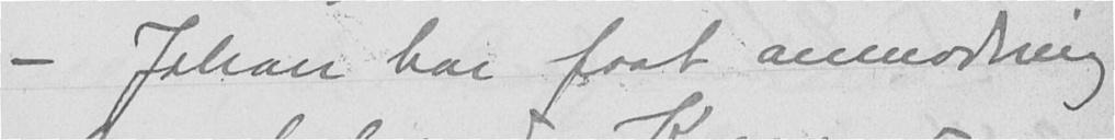- Johan har faat anmodning
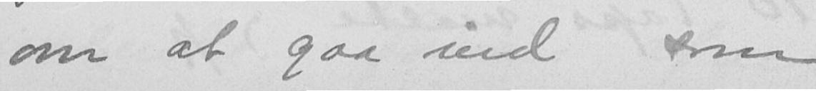om at gaa ind som
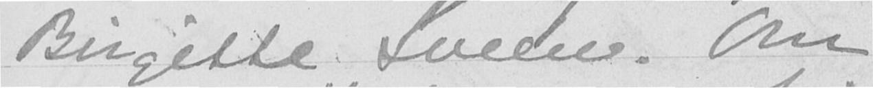Birgitte Sveen. Om
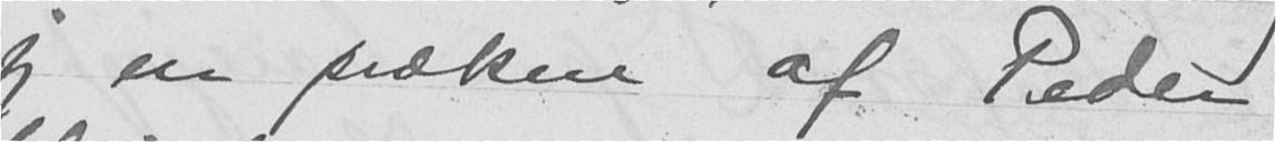jeg en præken af Peder
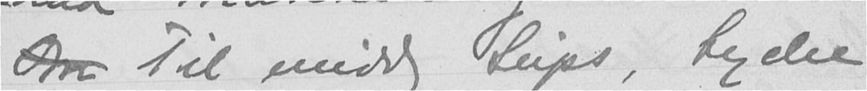Om Til middag Seips, Lyche
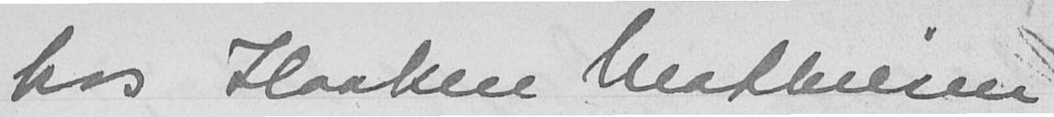hos Haaken Mathiesen
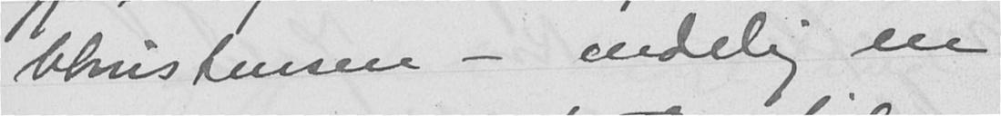Christensen - endelig en
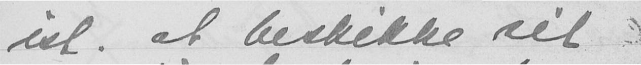ist. at beskikke sit
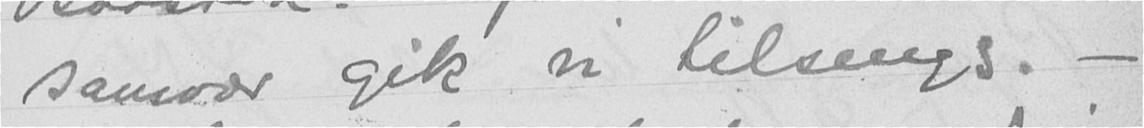samvær gik vi tilsengs. -
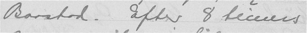Baastad. Efter 8 timers
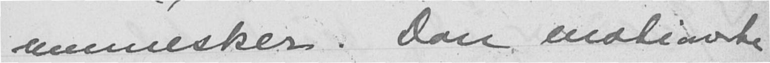mennesker. Don motionerte
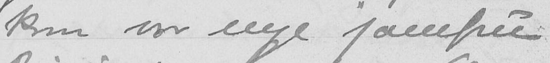kom vor nye jomfru
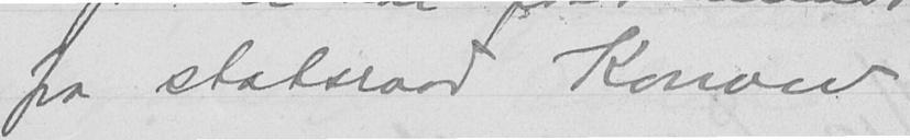fra statsraad Konow
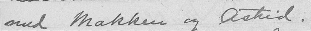med Makken og Astrid.
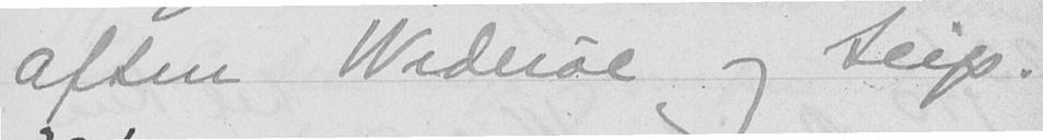aften Widerøe og Seip.
